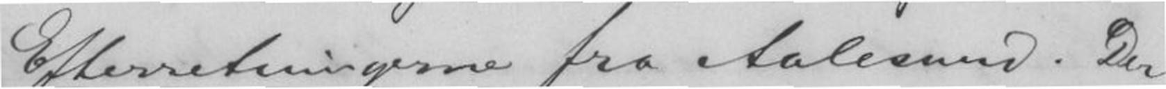Efterretningerne fra Aalesund. Der
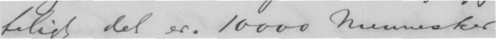teligt det er. 10000 Mennesker
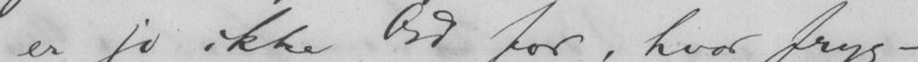er jo ikke Ord for, hvor fryg-
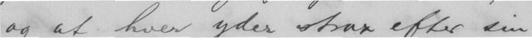og at hver yder strax efter sin
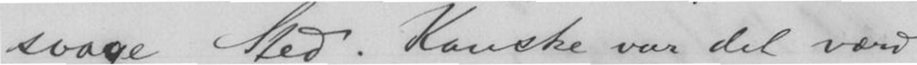svage Sted. Kanske var det værd
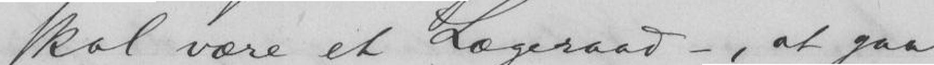skal være et Lægeraad -, at gaa
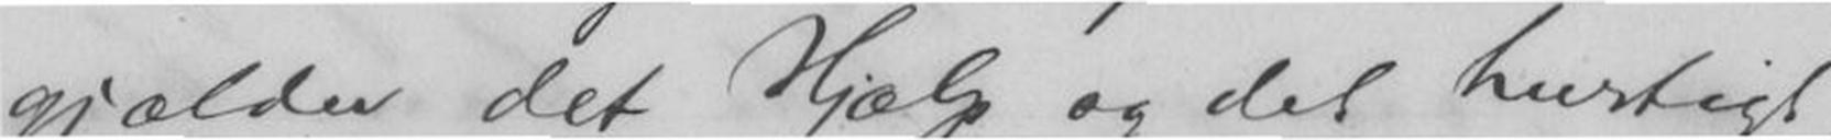gjælder det Hjælp og det hurtigt,
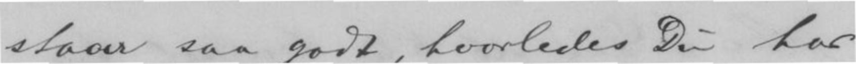staar saa godt, hvorledes Du har
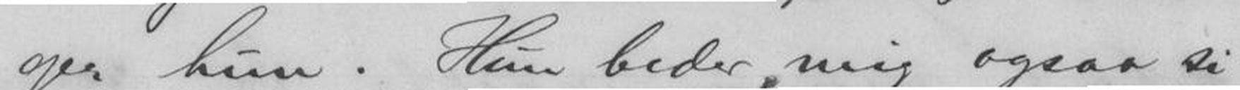ger hun. Hun beder mig ogsaa si-
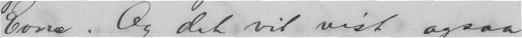Evne. Og det vil vist ogsaa
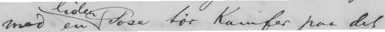med en liden Pose tør Kamfer paa det
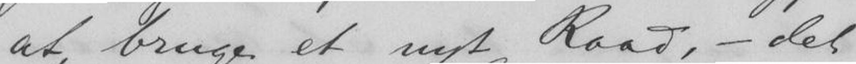at bruge et nyt Raad, - det
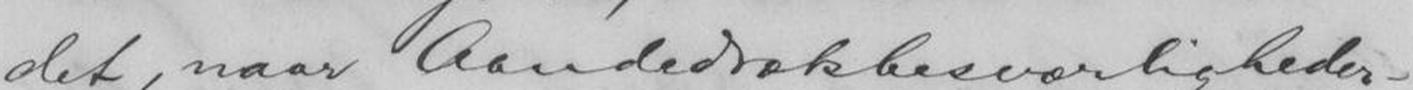det, naar Aandedrætsbesværligheder-
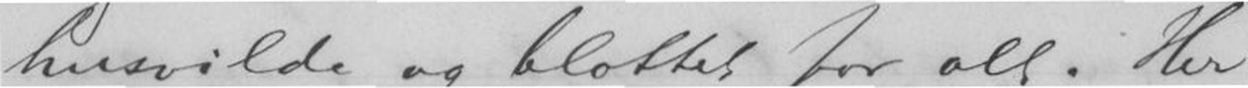husvilde og blottet for alt. Her
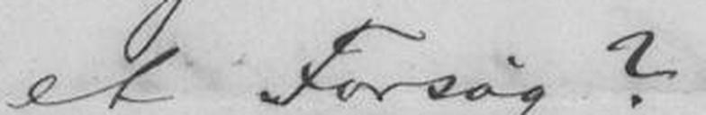et Forsøg?
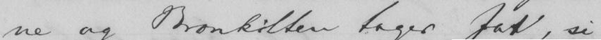ne og Bronkitten tager fat, si-
